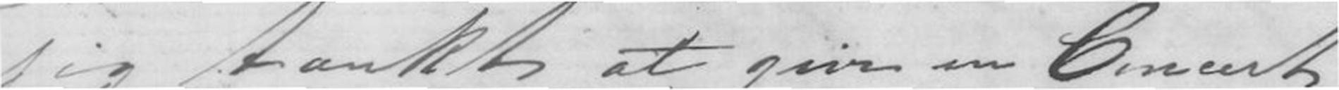jeg tænkt at give en Concdrt
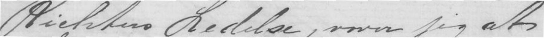Richters Ledelse, vover jeg at
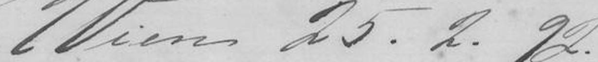Wien 25.2.92.
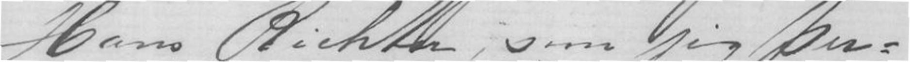Hans Richter, som jeg per-
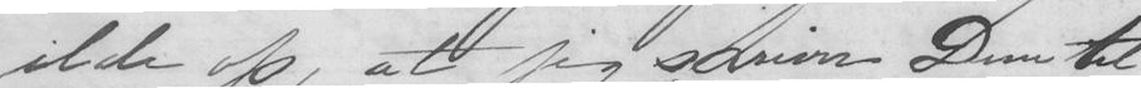ilde op, at jeg skriver Dem til !
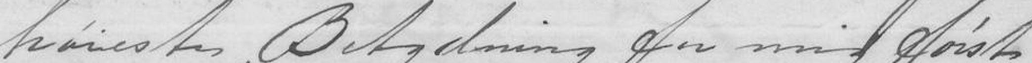høieste Betydning for mig først
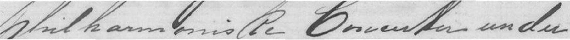philharmoniske Concerter under
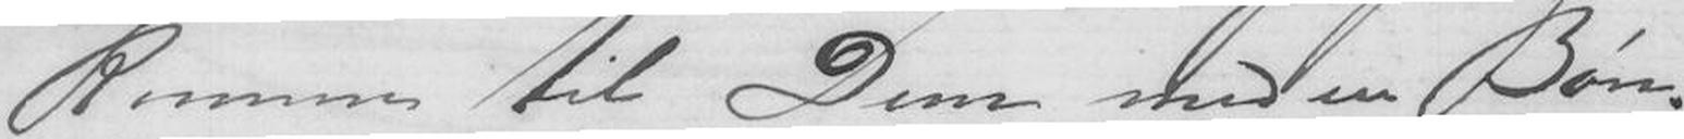komme til Dem med en Bøn.
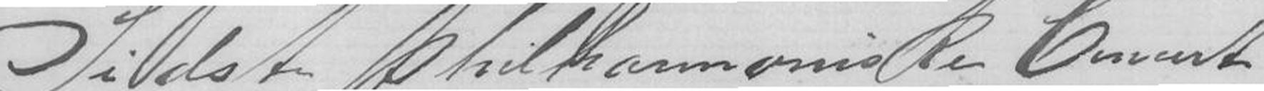Sidste philharmoniske Concert
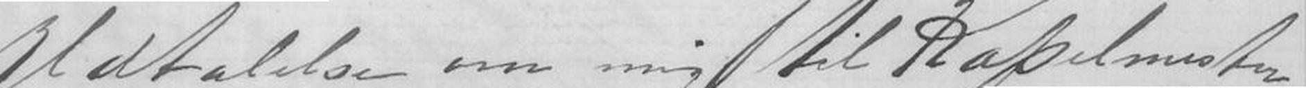Udtalelse om mig til Kapelmester
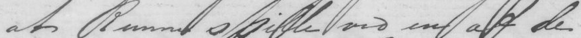at kunne spille ved en af de
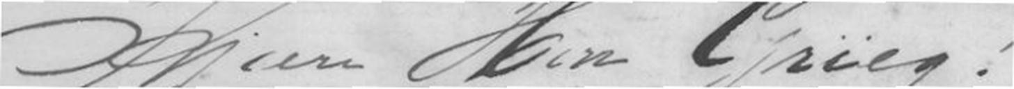Kjære Herr Grieg !
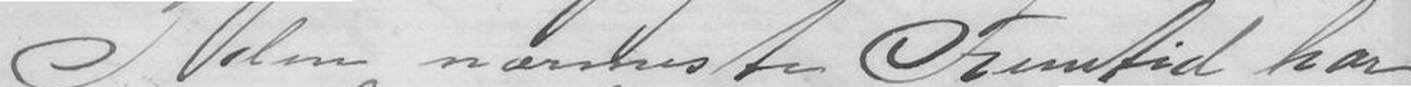I den nærmeste fremtid har
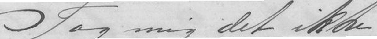Tag mig det ikke
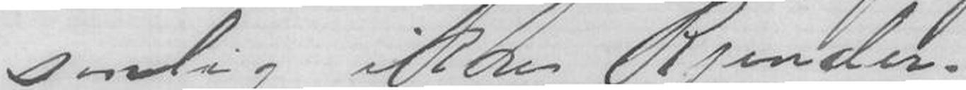sonlig ikke kjender.
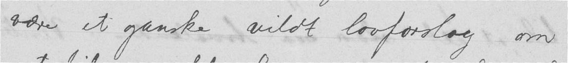være et ganske vildt lovforslag om
